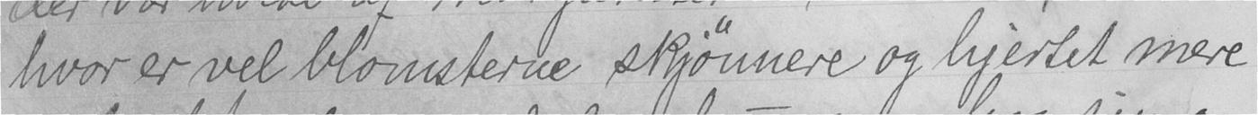hvor er vel blomsterne skjønnere og hjertet mere
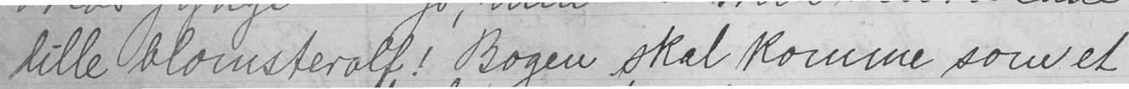lille blomsteralf! Bogen skal komme som et
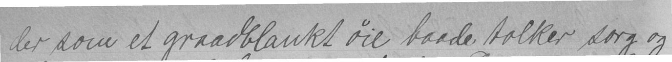der som et graadblankt øie baade tolker sorg og
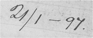21/1 - 97.
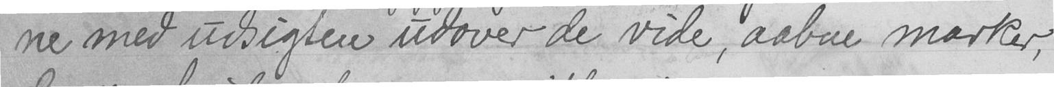ne med udsigten udover de vide, aabne marker,
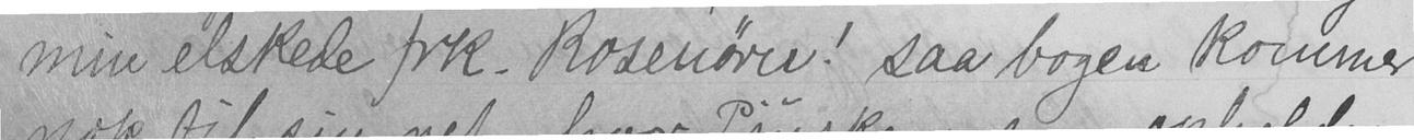min elskede frk. Rosenørn! saa bogen kommer
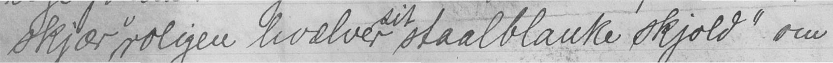skjær "roligen hvælver sit staalblanke skjold" om
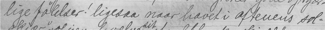lige følelser! ligesaa naar havet i aftenens sol-
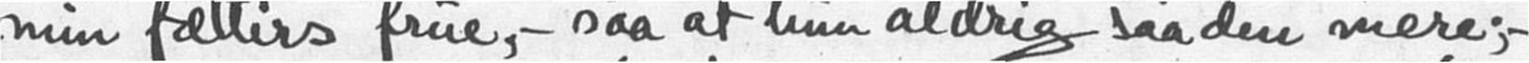min fætters frue, - saa at hun aldrig saa den mere;
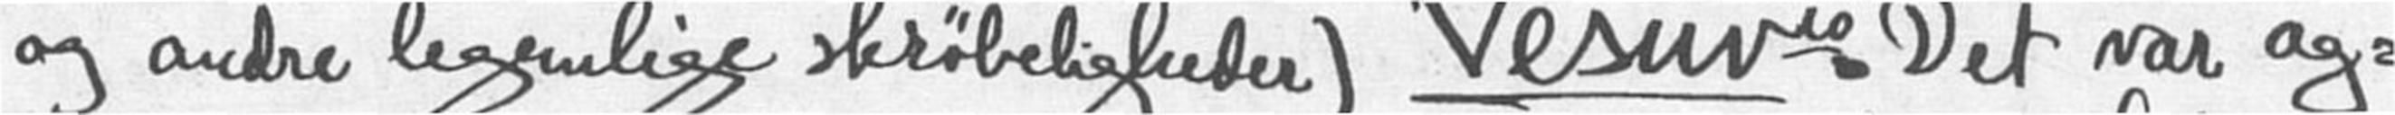og andre legemlige skrøbeligheder) Vesuvio. Det var og-
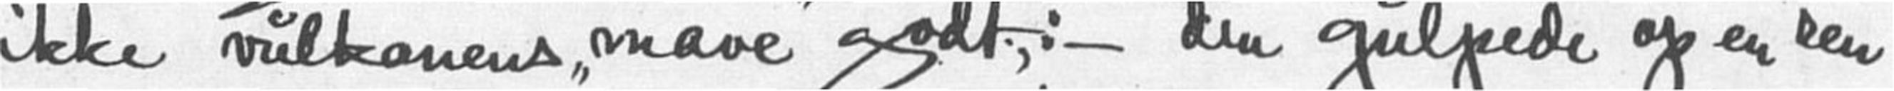ikke vulkanens "mave" godt, : - den gulpende op en ren
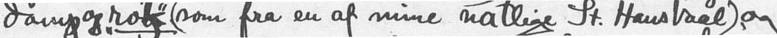damp og "røk" "(som fra en af mine natlige St. Hansbaal), og
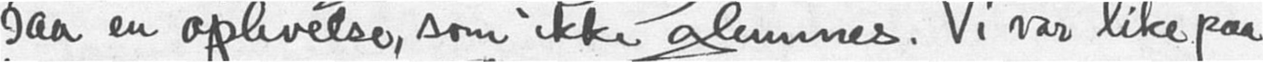saa en oplevelse, som ikke glemmes. Vi var like paa
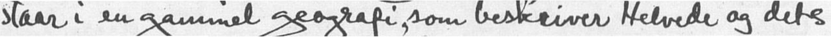staar i en gammel geografi, som beskrive Helvede og dets
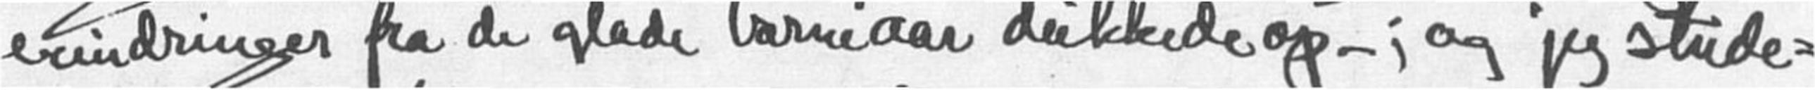erindringer fra de glade barneaar dukkede op -; og jeg stude-
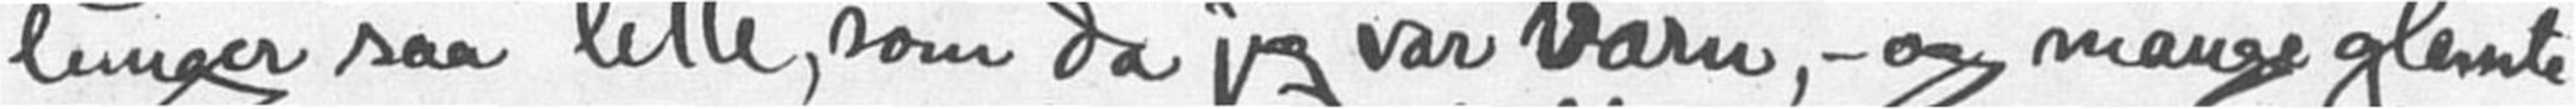lunger saa lette, som da jeg var barn,- og mange glemte
